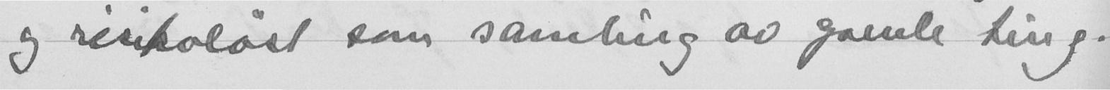og risikoløst som samling av gamle ting.
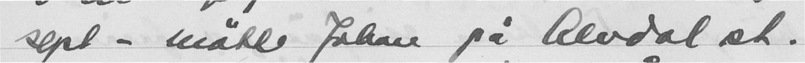sept - møtte Johan på Alvdal st.
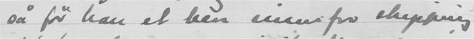så får han et ben innenfor skipping
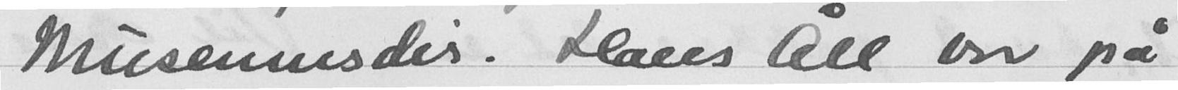Museumsdirekt. Hans Åll var på
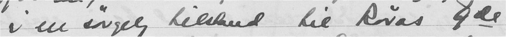i en sørgelig tilstand til Røros 9de
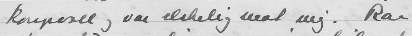Kongsvoll og var elskelig mot mig. Rar
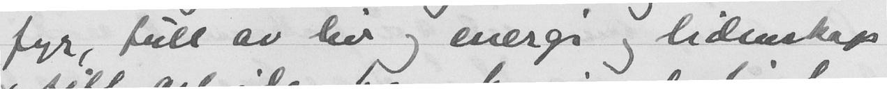fyr, full av liv og energi og lidenskap
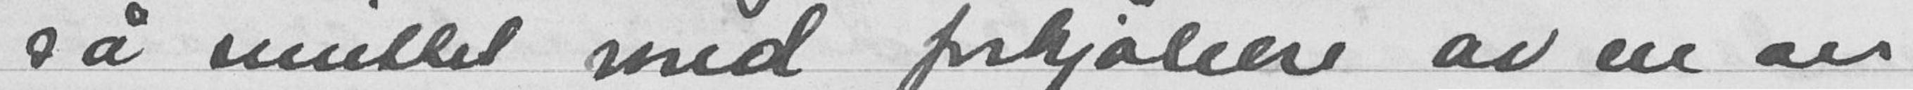så smittet med forkjølelse av en av
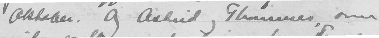Oktober. Og Astrid og Thommes, som
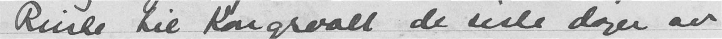Reiste til Kongsvoll de siste dager av
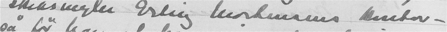skibsmegler Erling Mortensens kontor -
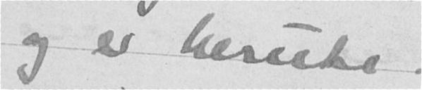og er herute.
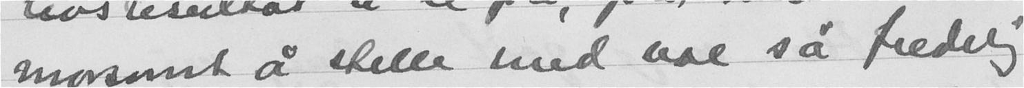morsomt å stille med noe så fredelig
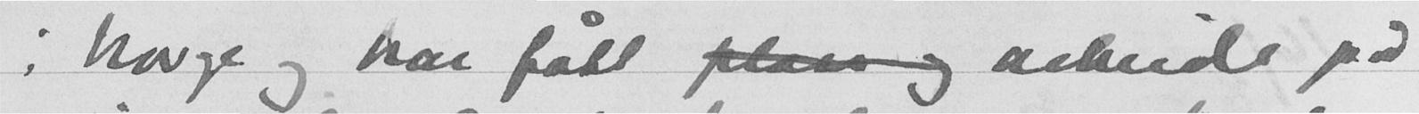i Norge og har fått  arbeide på
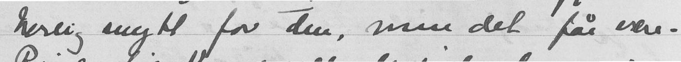herlig snytt for den, men det får være.
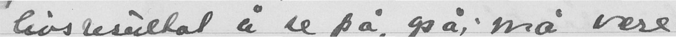livsresultat å se på, også, må være
